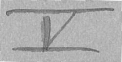V
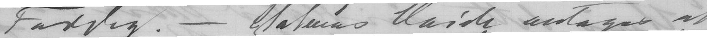Fødeby. - Statuens Høide antages at
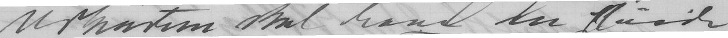Udkastene skal have en Høide
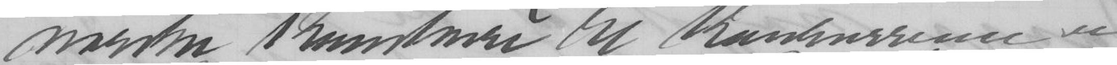norske kunstnere til Konkurrance om
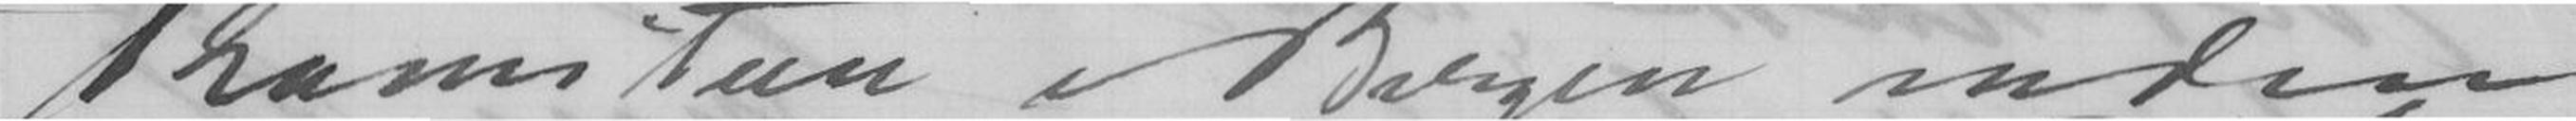til Komiteen i Bergen inden
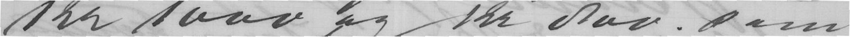Kr 1000 og Kr 800. Som
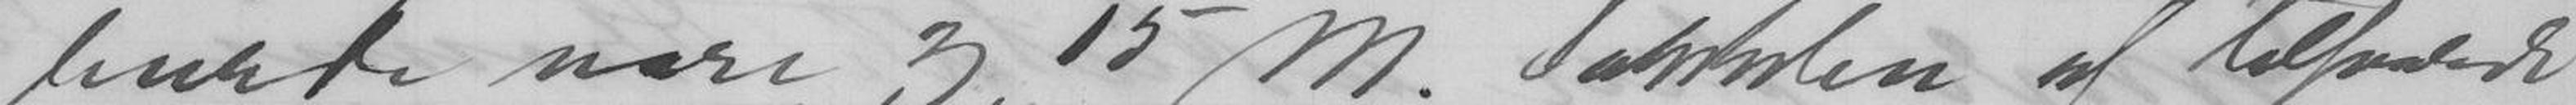burde være 3,15 M. Sokkelen af tilsvarede
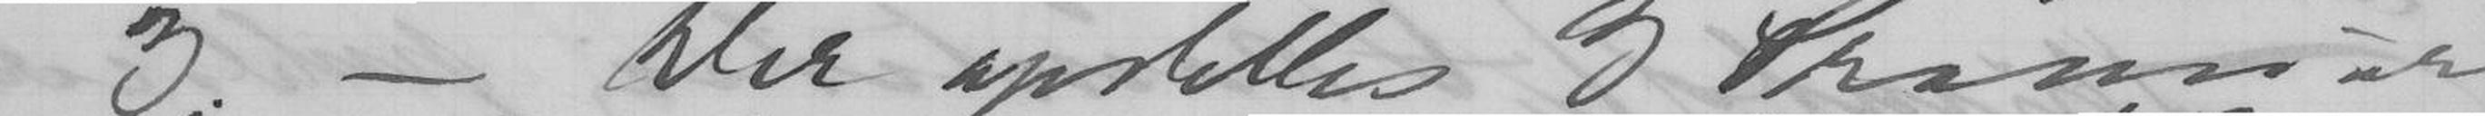3. - Der opstilles 3 Premier
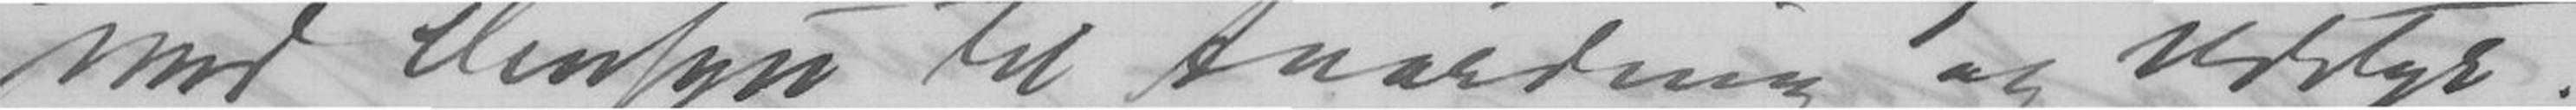med Hensyn til Anordning og Udstyr.
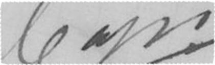Copi
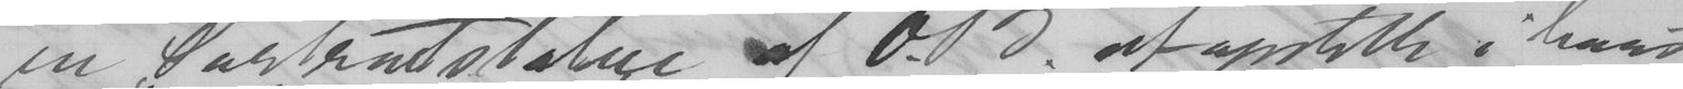en Portrætstatue af O. B. at opstille i hans
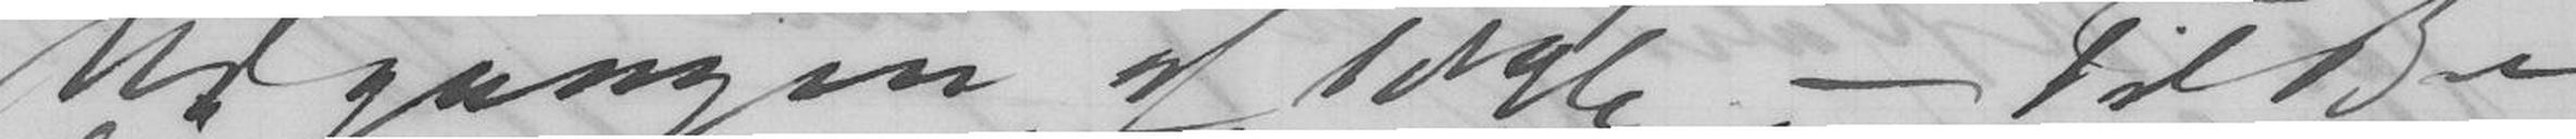Udgangen af 1896 - Til Be-
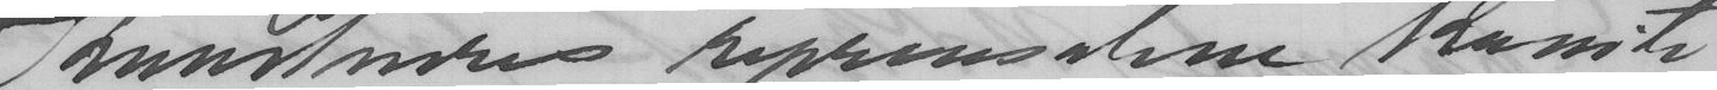Kunstneres reprensabne Komite
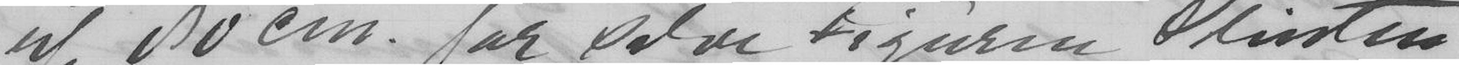af 80 cm. for selve Figuren, Plinten
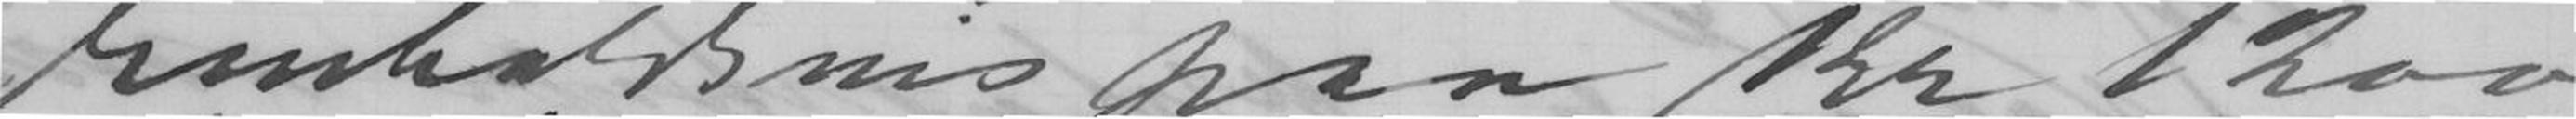henholdsvis paa Kr 1200
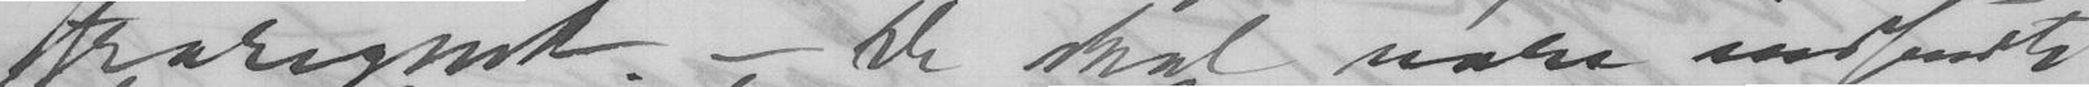fraregnet. - De skal være indsendte
